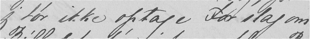jeg tør ikke optage Forslag om
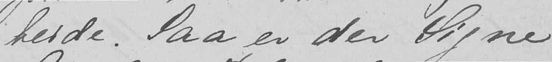beide. Saa er der Signe
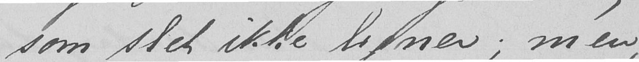som slet ikke ligner; men
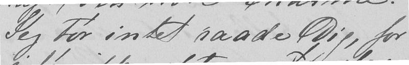Jeg tør intet raade Dig, for
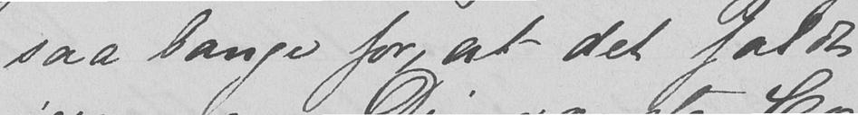saa bange for, at det faldt
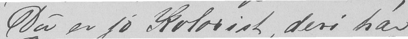Du er jo Kolorist, deri har
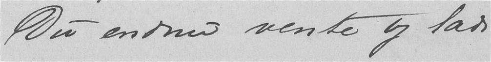Du endnu vente og lade
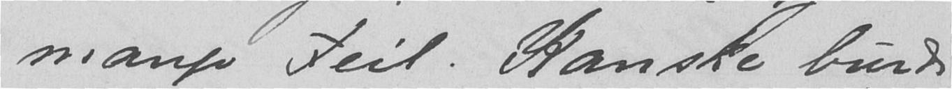mange Feil. Kanske burde
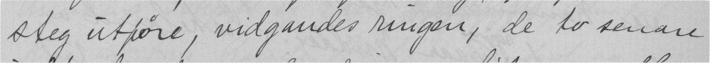steg utføre, vidgandes ringen, de to senare
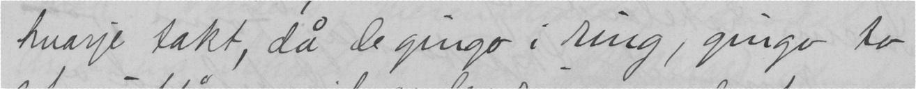hvarje takt, då de gingo i ring, gingo to
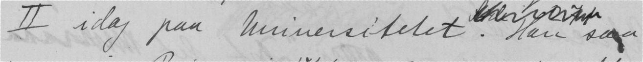II idag paa Universitetet. Han saa
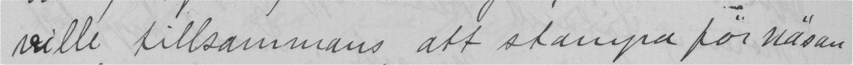ville tillsammans att stampa før näsan
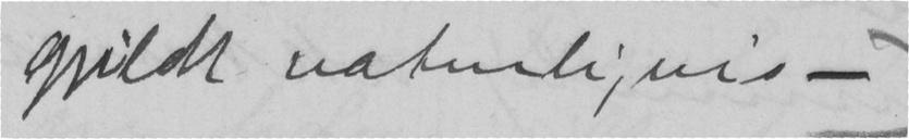gjildt naturligvis -
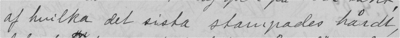af hvilka det sista stampades hårdt,
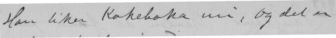Han liker Kokeboka mi, og det er
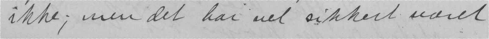ikke; men det har vel sikkert været
